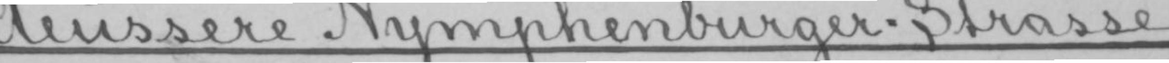Aeussere Nymphenburger-Strasse
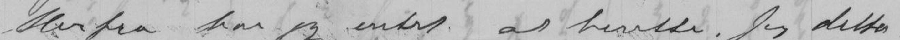Herfra har jeg intet at berette. Jeg delta-
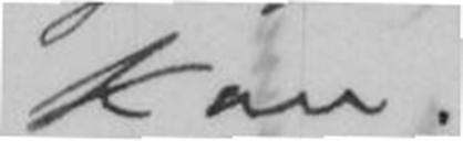kan.
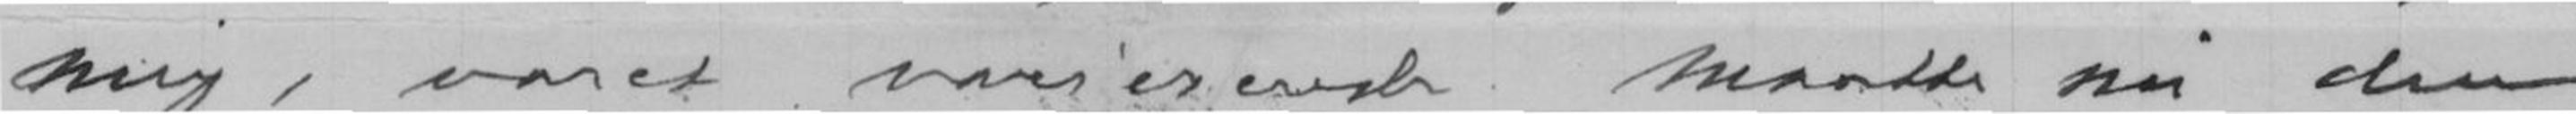mig, varet varierede. Maatte nu den
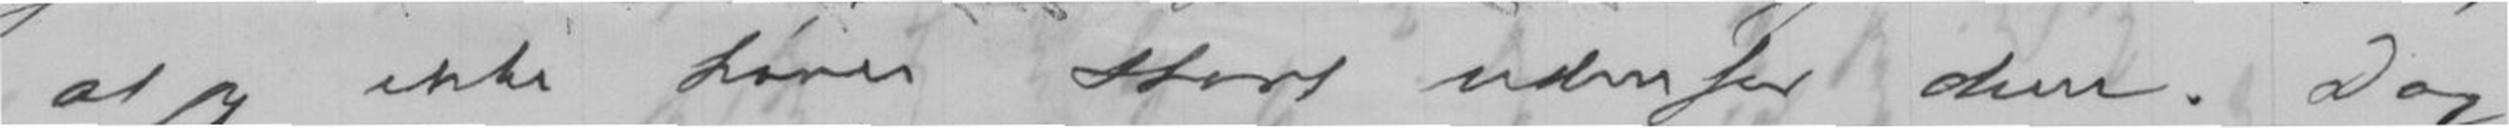at jeg ikke hører stort udenfor dem. Dog
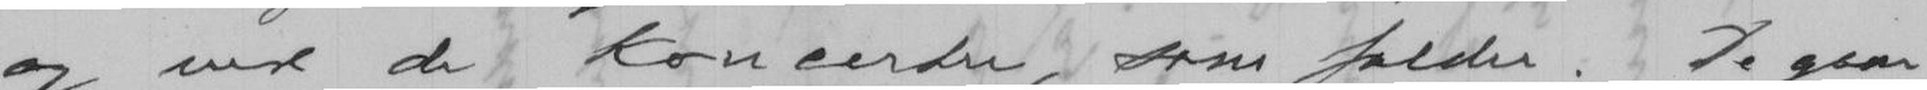og ved de koncerter, som falder. De gaar
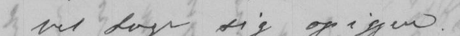vil tage sig op igjen.
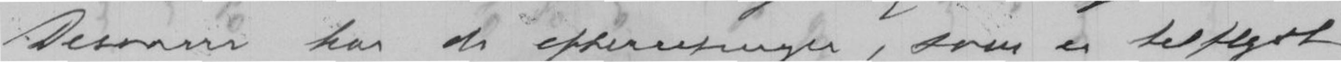Desværre har de efterretninger, som er tilflydt
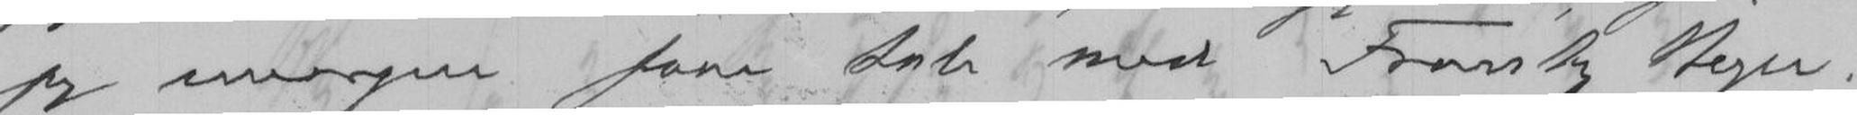jeg imorgen faar tale med Frantz Beyer.
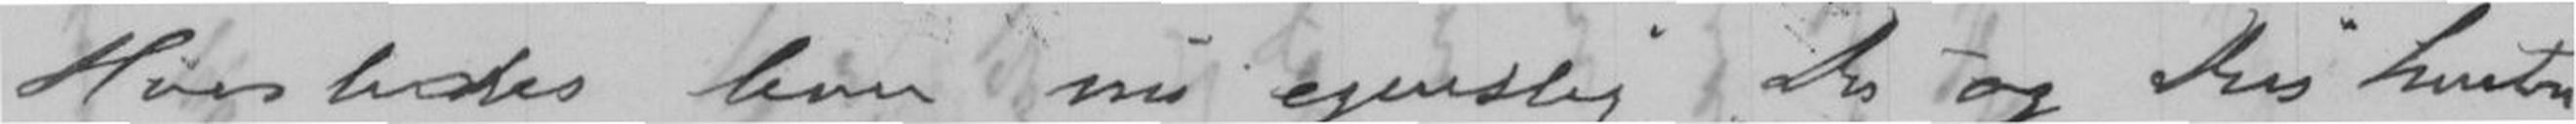Hvorledes lever nu egentlig Du og Din hustru.
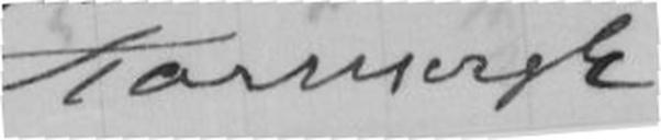Karl Bergh
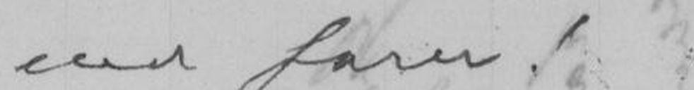end farer!
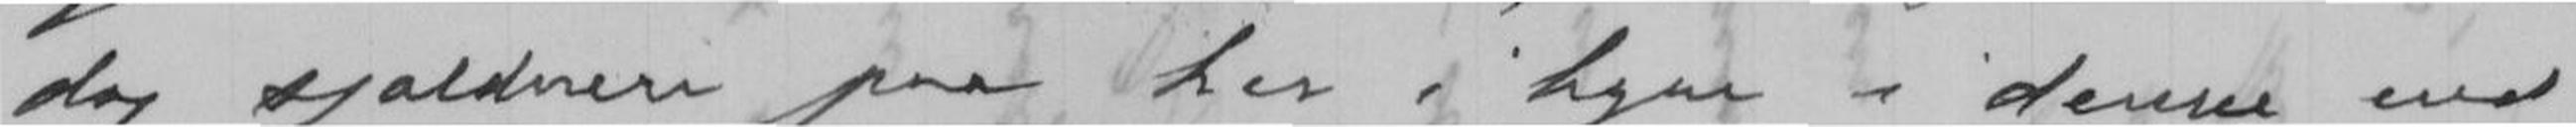dog sjældnere paa her i byen i denne end i
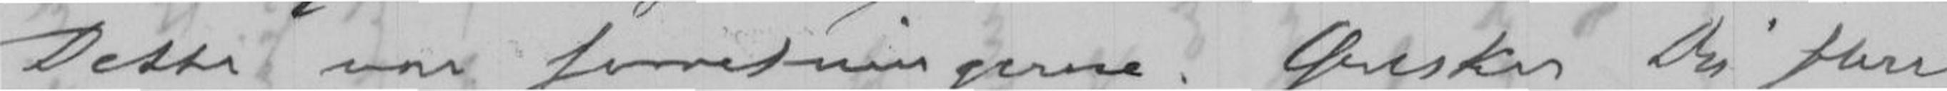Dette var forretningerne. Ønsker Du flere
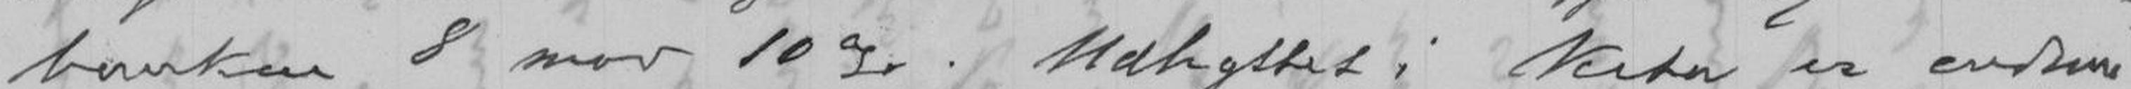banken 8 mod 10%. Udbyttet i Vesta er endnu
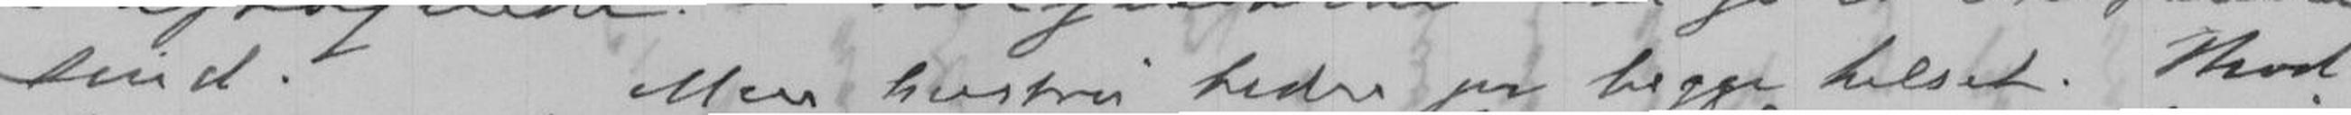sind. Min hustru beder jer begge tilset. Mod-
